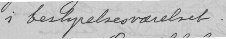i bestyrelsesværelset.
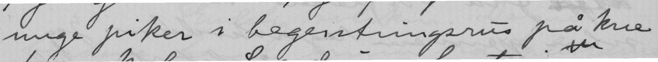unge piker i begeistringsrus på kne
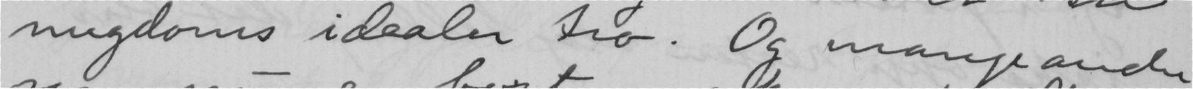ungdoms idealer tro. Og mange andre
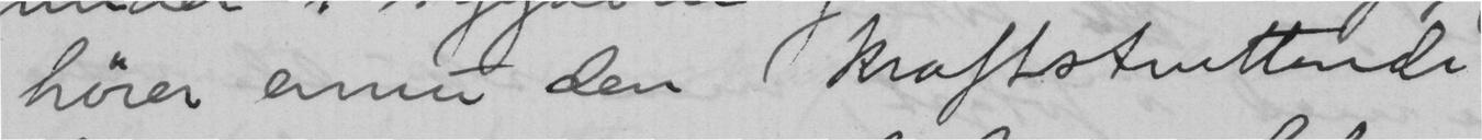hører ennu den kraftstrettende
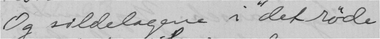Og sildelagene i "det røde
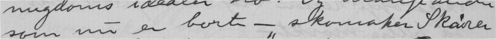som nu er borte - skomaker Skårer
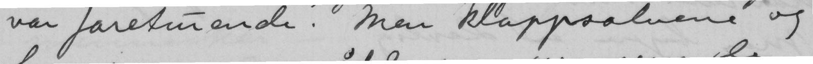var faretruende. Men klappsalvene og
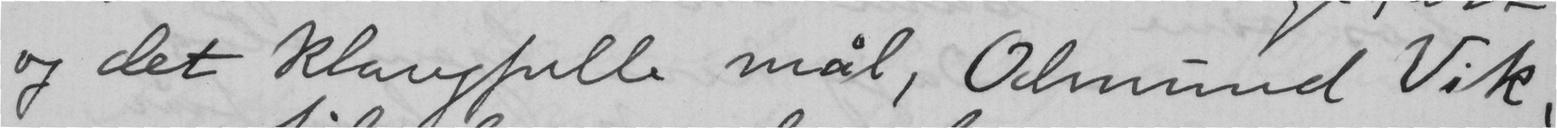og det klangfulle mål, Odmund Vik,
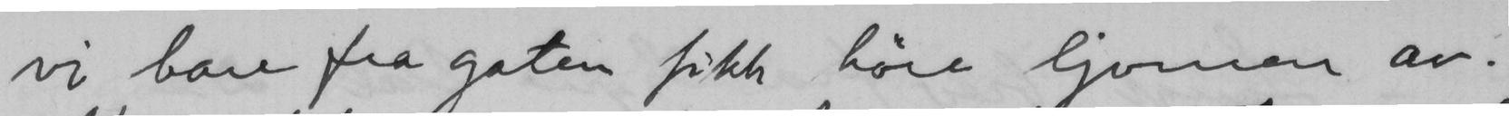vi bare fra gaten fikk høre ljornen av.
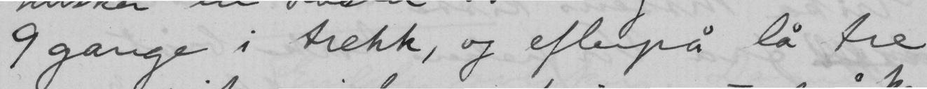9 gange i trekk, og efterpå lå tre
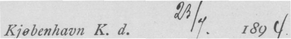Kjøbenhavn K. d. 23/7 1894
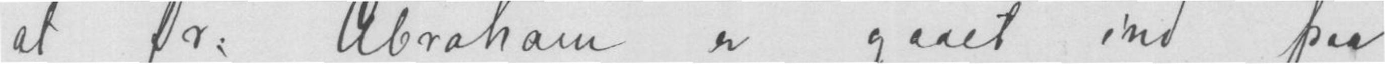at Dr, Abraham er gaaet ind paa,
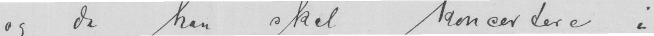og de han skal koncertere i
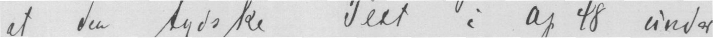at den tydske Text i op 48 under-
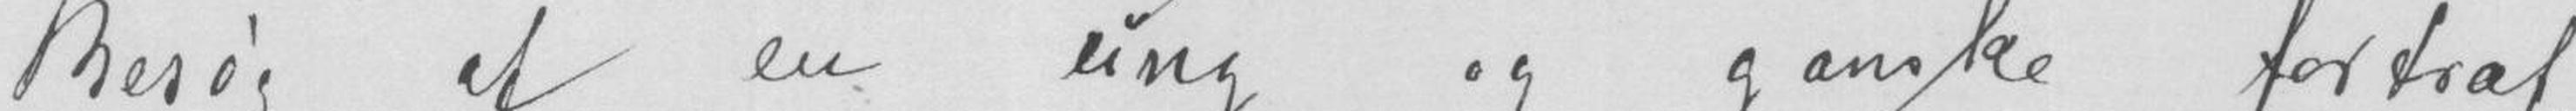Besøg af en ung og ganske fortræf-
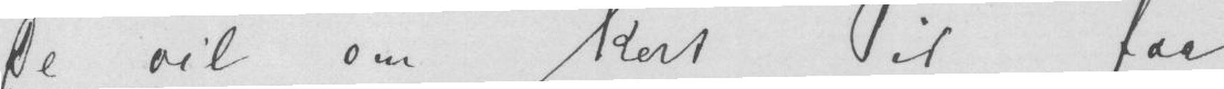De vil om kort Tid faa
